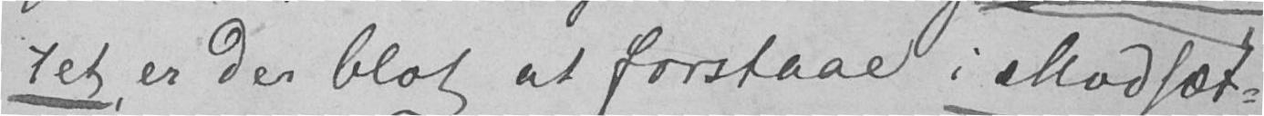tet er der blot at forstaae i Modsæt-
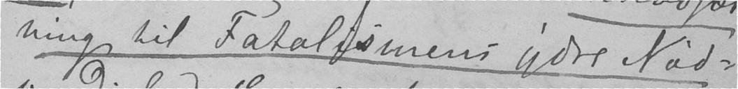ning til Fatalismens ydre Nød-
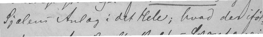Sjælens Anlæg i det Hele; hvad der iføl-
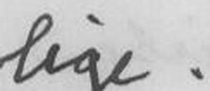lige.
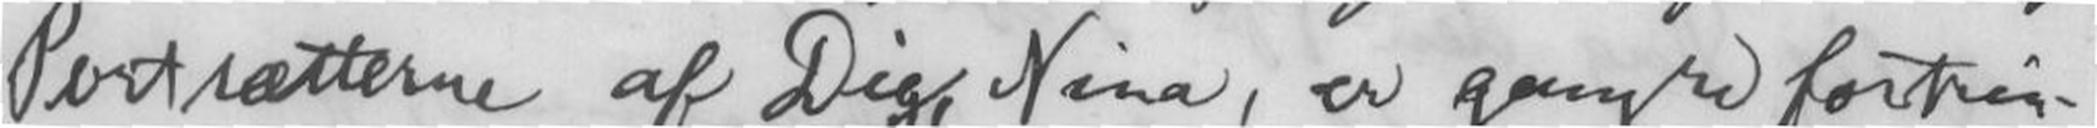Portrætterne af Dig, Nina, er ganske fortin-
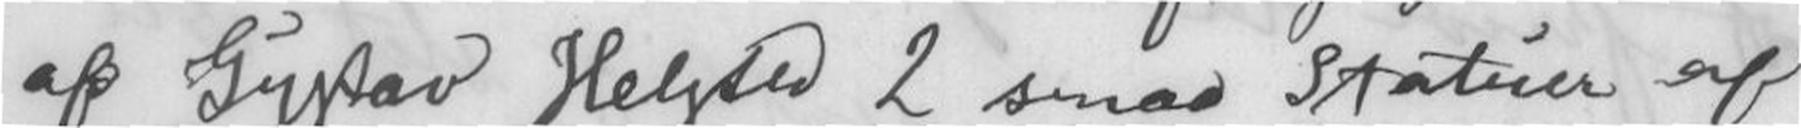af Gustav Helsted 2 smaa Statuer af
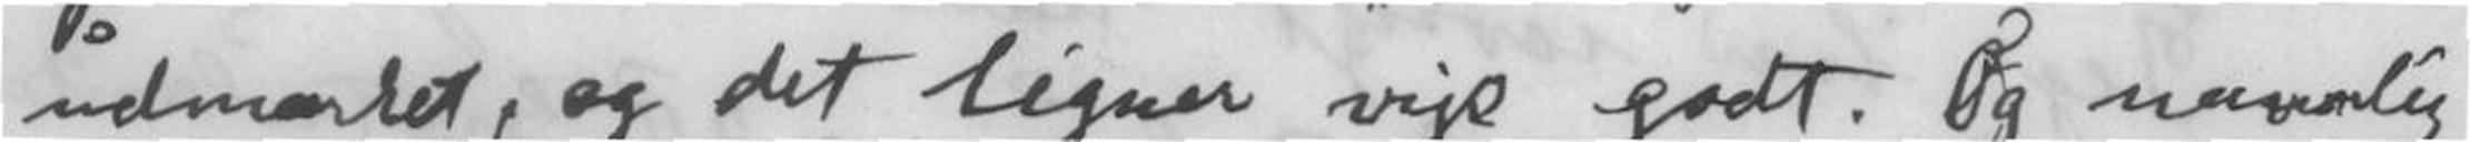udmærket, og det ligner vist godt. Og navnlig
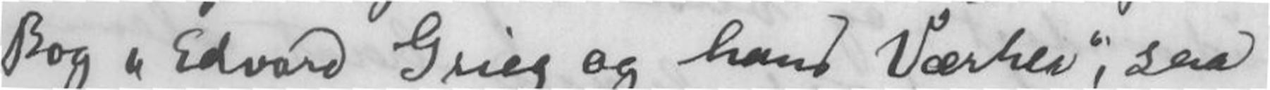Bog Edvard Grieg og hans Værker, saa
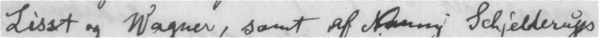Liszt og Wagner, samt af Nanny Schjelderups
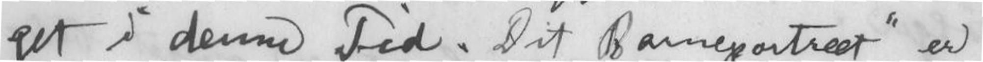get i denne Tid. Dit Barneprotræt er
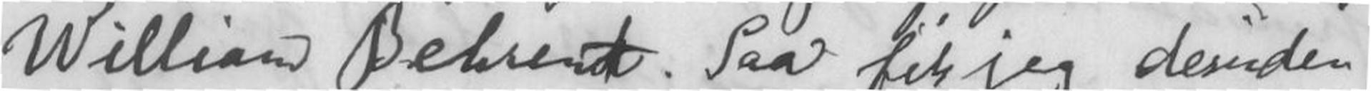William Behrent. Saa fik jeg desuden
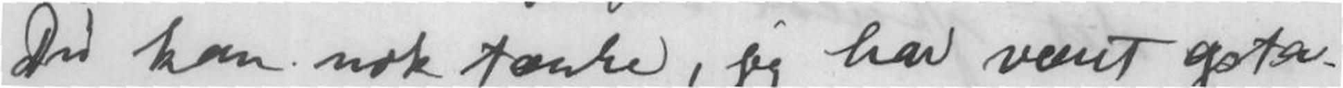Du kan nok tænke, jeg har været opta-
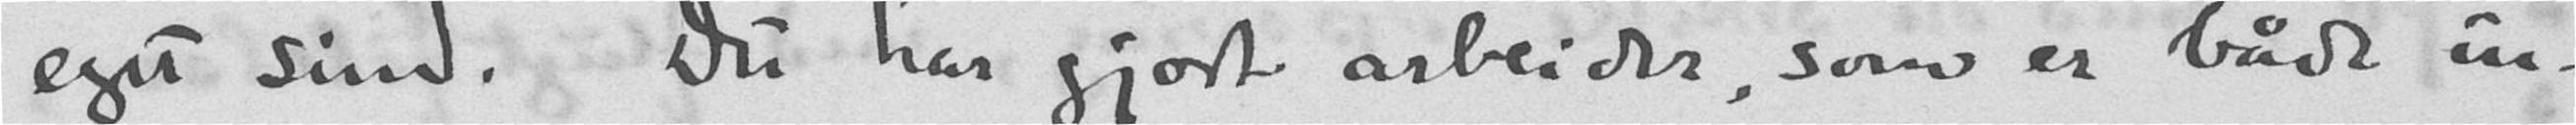eget sind. Du har gjort arbeider, som er både in-
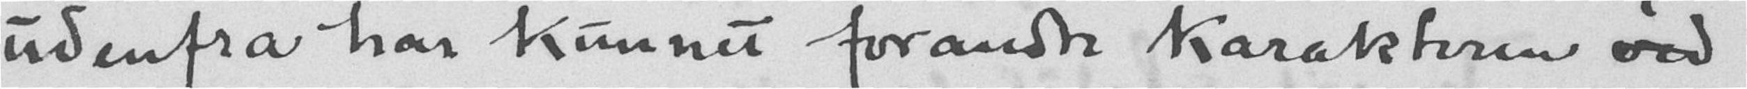udenfra har kunnet forandre karakteren ved
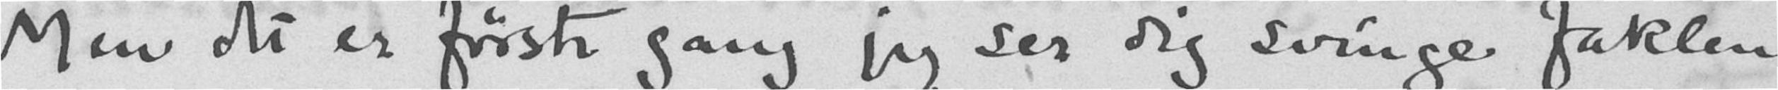Men det er første gang jeg ser dig svinge faklen
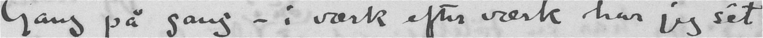Gang på gang - i værk efter værk har jeg set
